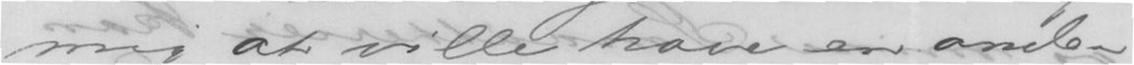mig at ville have en anden,
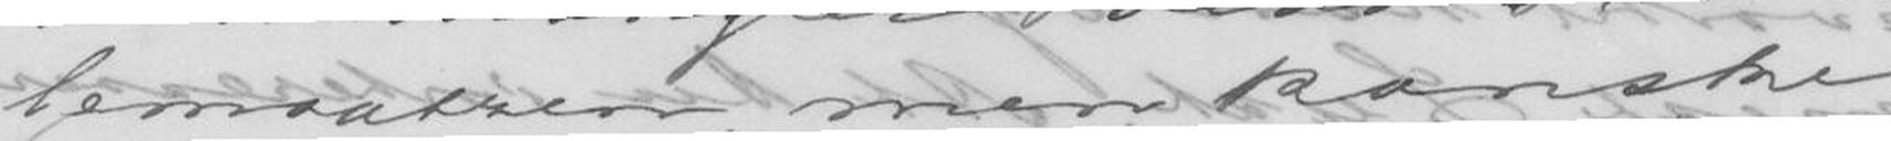lemsatsen, men kanske
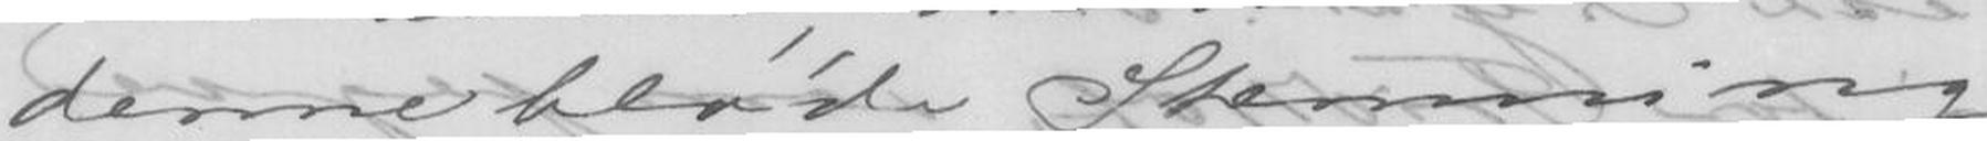denne bløde Stemning
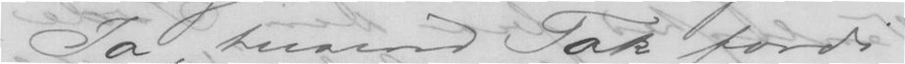-Ja, tusind Tak fordi
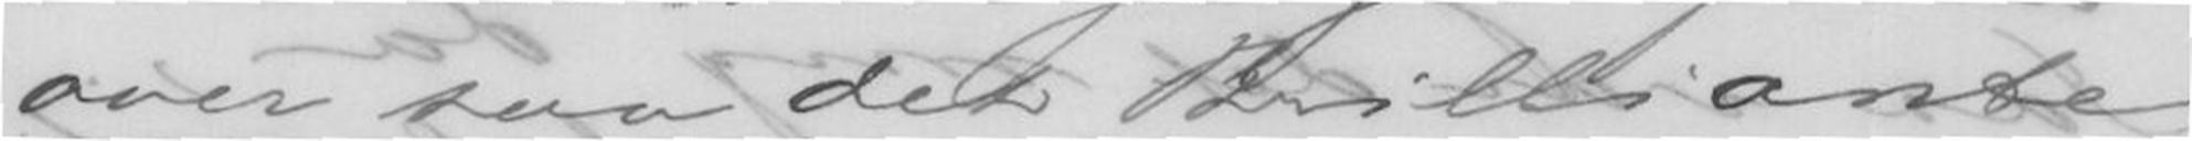over, saa det Brilliante
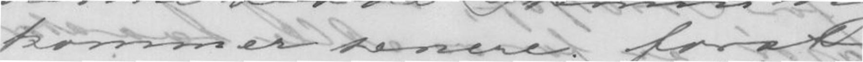kommer senere; forat
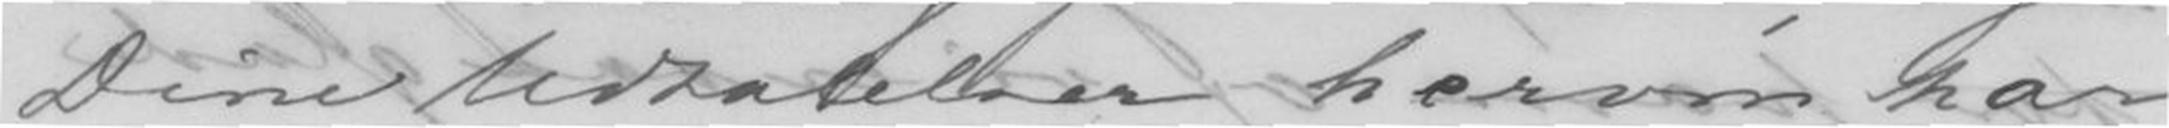Dine Udtalelser herom har
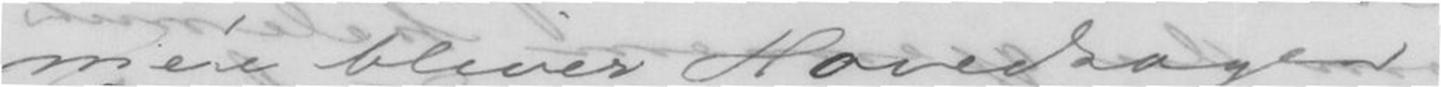mere bliver Hovedsagen,
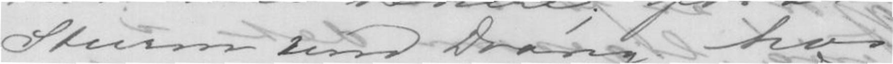Sturm und Drang hos
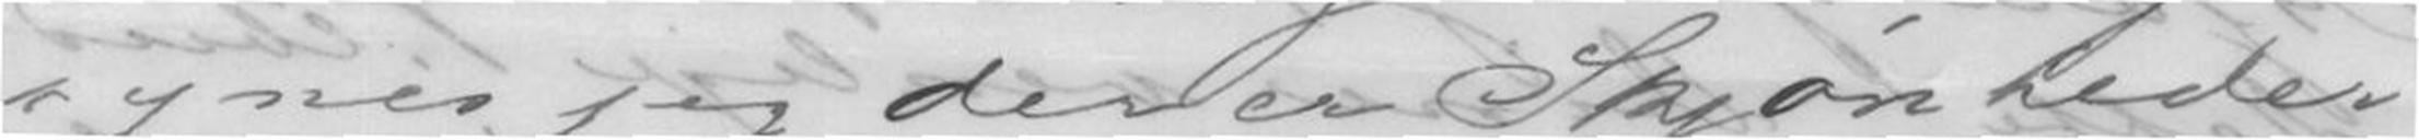synes jeg der er Skjønheder
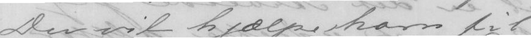Du vil hjælpe ham til
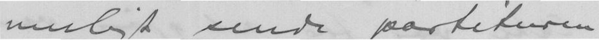muligt sende partituren
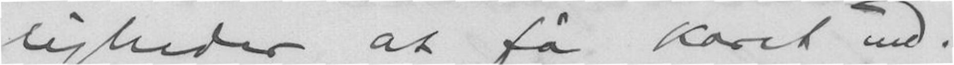ligheder at få koret ind-
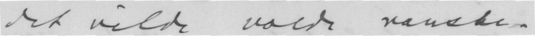det vilde volde vanske-
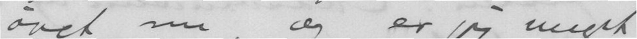øvet nu, og er jeg meget
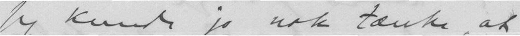Jeg kunde nok tænke, at
